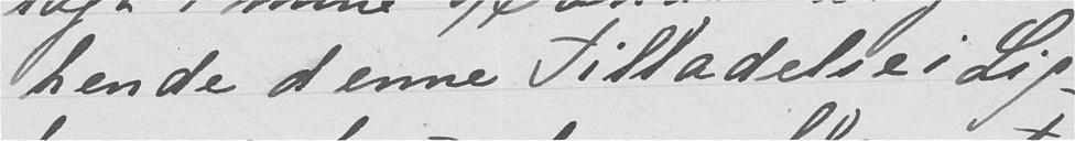hende denne Tilladelse i Lig-
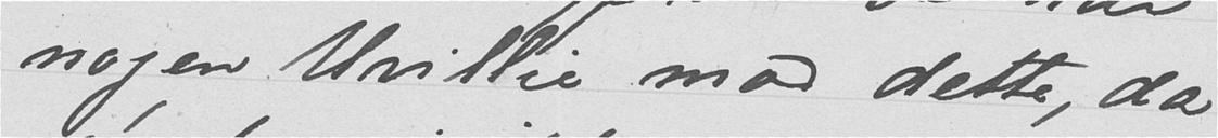nogen Uvillie mod dette, da
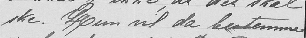ske. Hun vil da bestemme
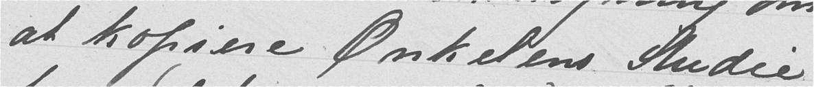at kopiere Onkelens Studie-
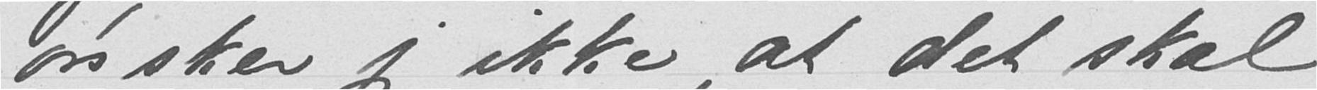ønsker jeg ikke, at det skal
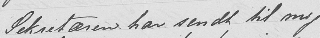Sekretæren har sendt til mig
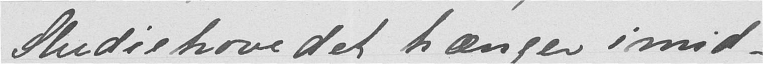Studiehovedet hænger imid-
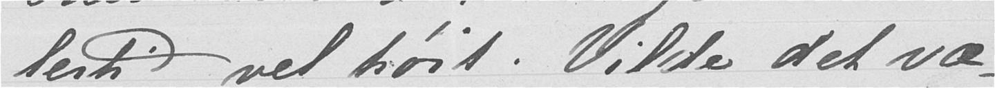lertid vel høit. Vilde det væ-
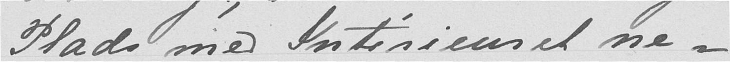Plads med Intérieuret ne-
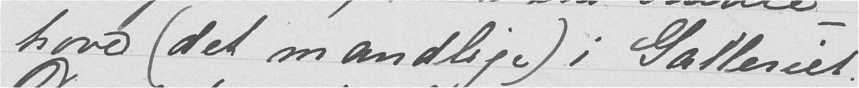hoved (det mandlige) i Galleriet.
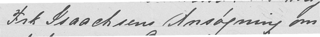Frk Isaachsens Ansøgning om
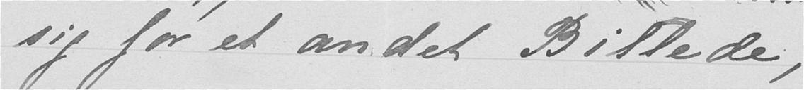sig for et andet Billede,
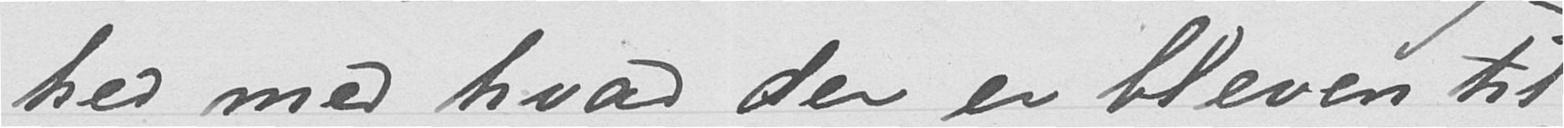hed med hvad der er bleven til-
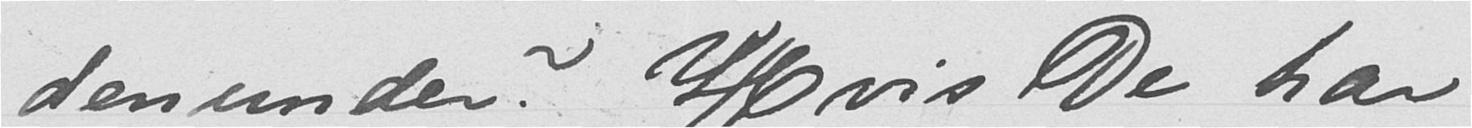denunder? Hvis De har
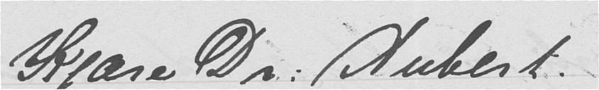Kjære Dr Aubert.
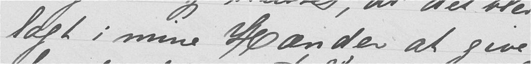lagt i mine Hænder at give
